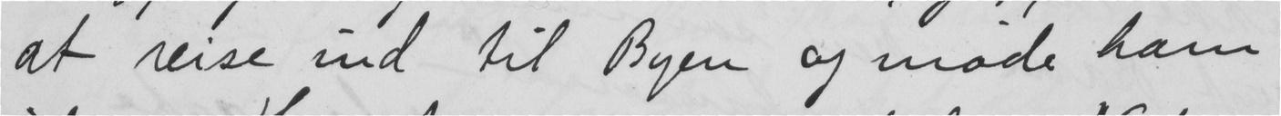at reise ind til Byen og møde ham
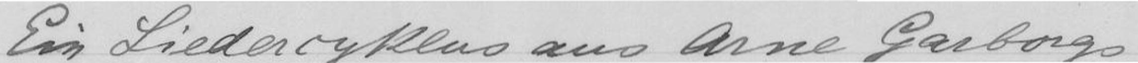Ein Liedercyklus aus Arne Garborgs
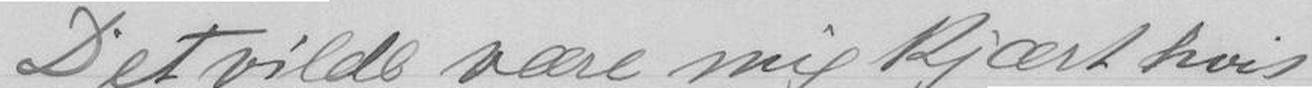Det vilde være mig kjært hvis
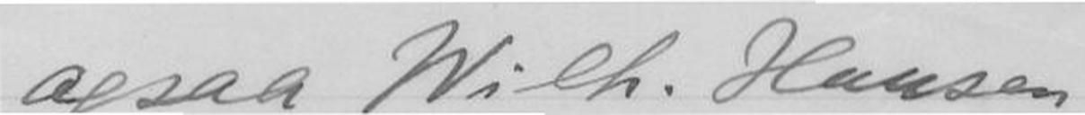ogsaa Wilh. Hansen
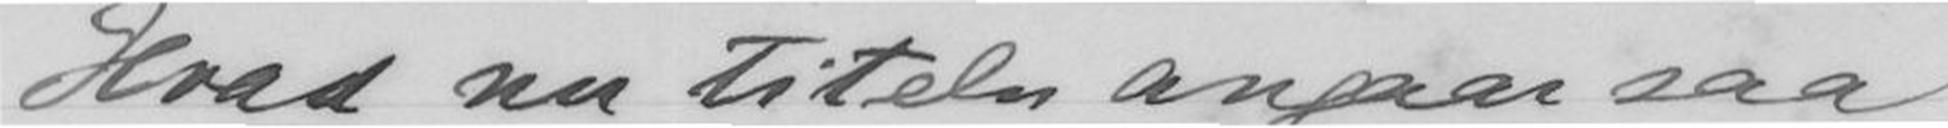Hvad nu tileln angaar saa
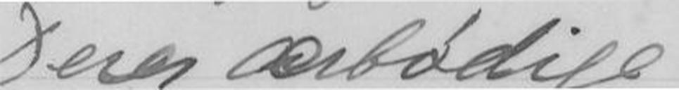Deres ærbødige
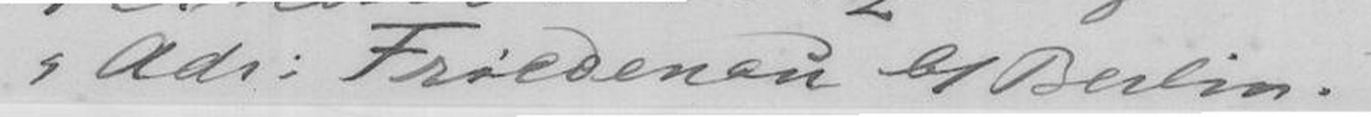"Adr: Friedenau b/Berlin.
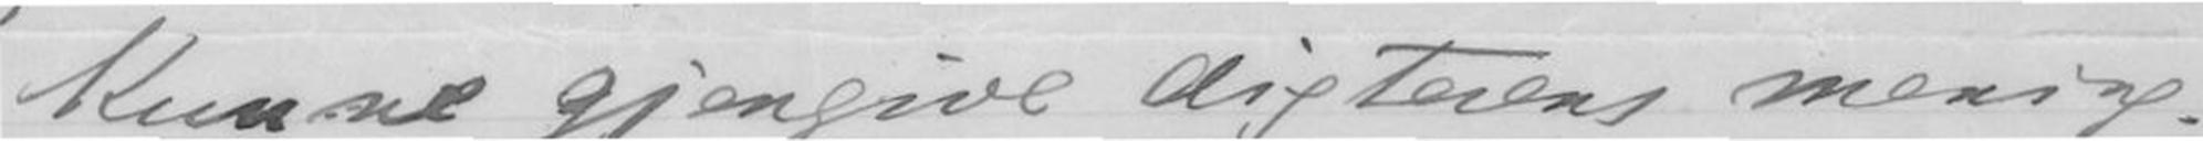kunne gjengive digterens mening.
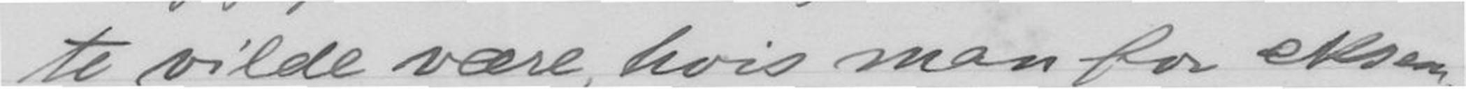te vilde være, hvis man for eksem-
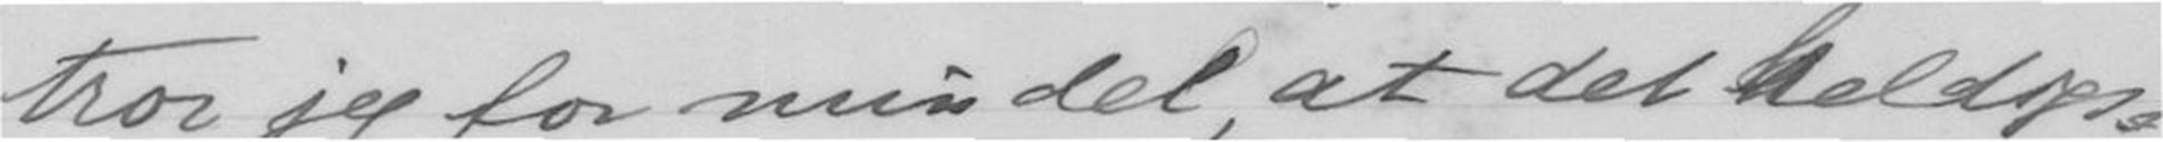tror jeg for min del, at det heldigs-
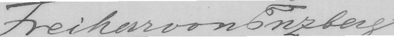Freiherr von Enzberg
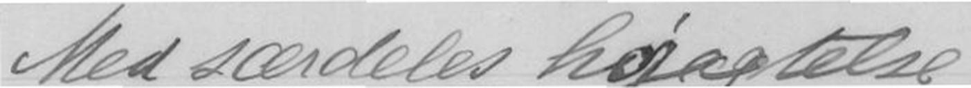Med særdeles højagtelse
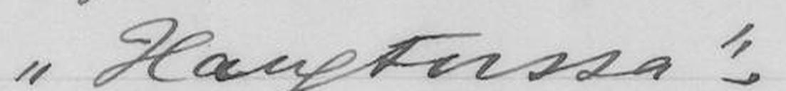"Haugtussa".
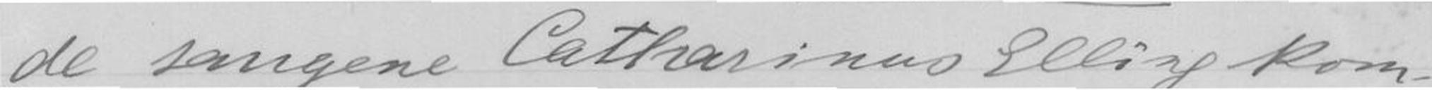de sangene Catharinus Elling kom-
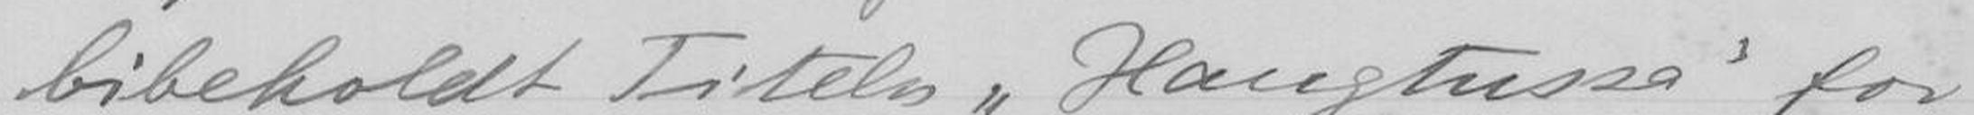bibelholdt titeln "Haugtussa" for
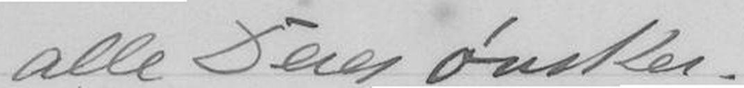alle Deres ønske.
